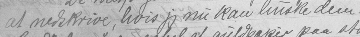at nedskrive, hvis jeg nu kan huske dem.
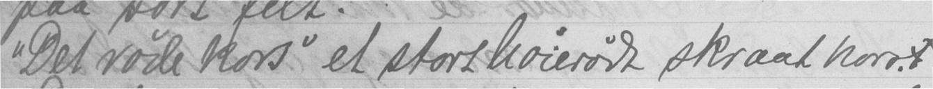"Det røde kors" et stort høierødt skraat korst.
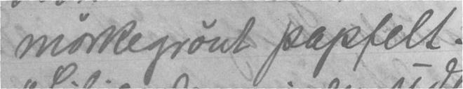mørkegrønt papfelt.
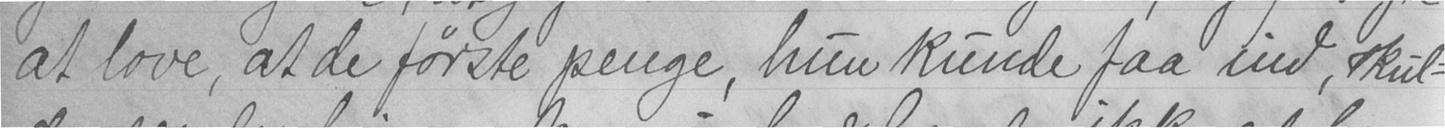at love, at de første penge, hun kunde faa ind, skul-
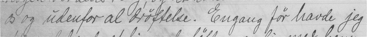os og udenfor al drøftelse. Engang før havde jeg
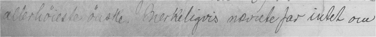allerhøieste ønske. Merkeligvis nævnte far intet om
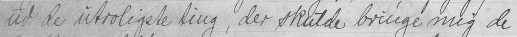ud de utroligste ting, der skulde bringe mig de
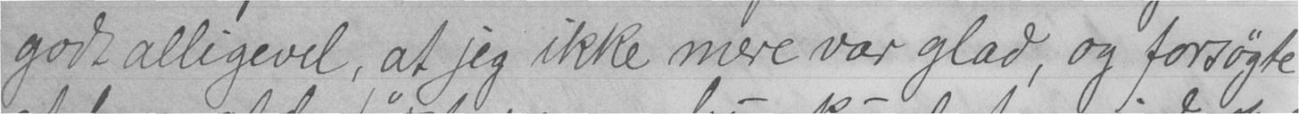godt alligevel, at jeg ikke mere var glad, og forsøgte
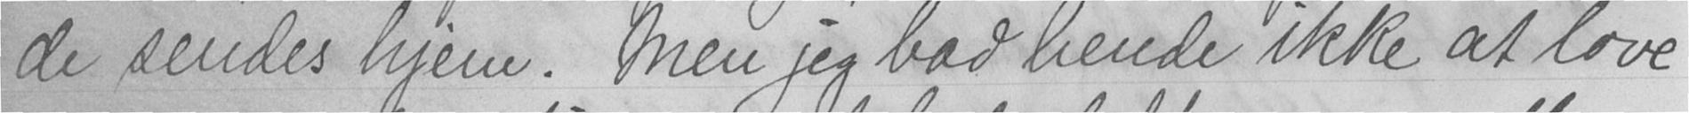de sendes hjem. Men jeg bad hende ikke at love
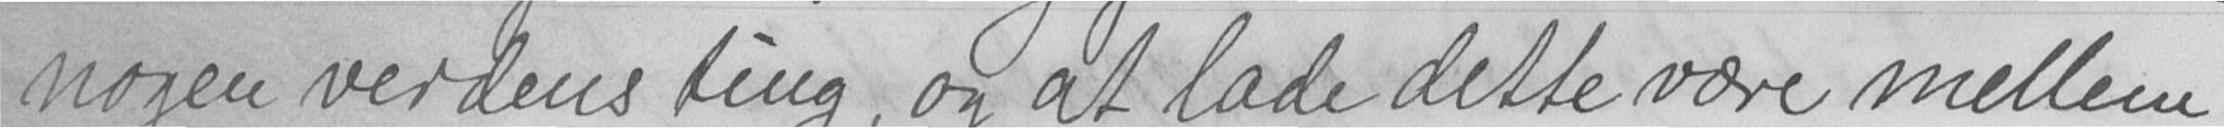nogen verdens ting, og at lade dette være mellem
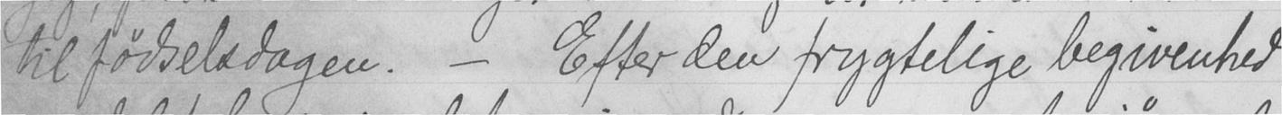til fødselsdagen. - Efter den frygtelige begivenhed
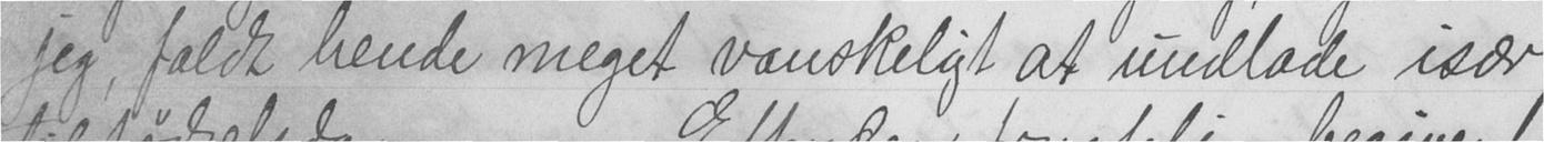jeg, faldt hende meget vanskeligt at undlade især
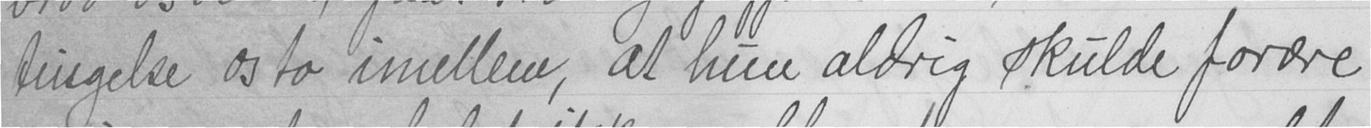tingelse os to imellem, at hun aldrig skulde forære
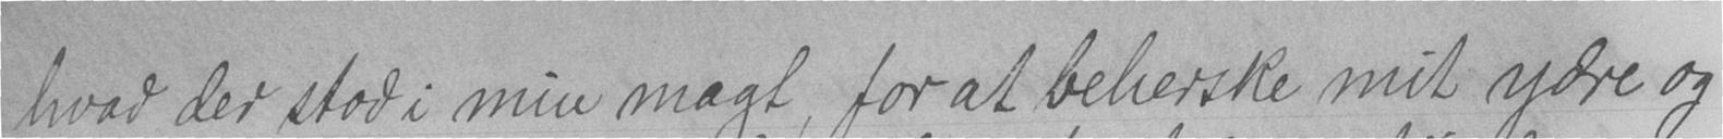hvad der stod i min magt, for at beherske mit ydre og
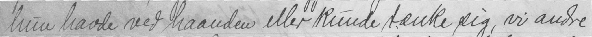hun havde ved haanden eller kunde tænke sig, vi andre
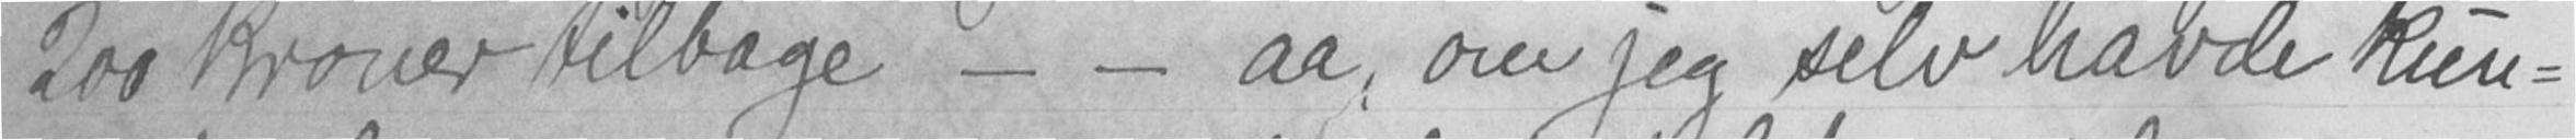200 kroner tilbage - - aa, om jeg selv havde kun-
